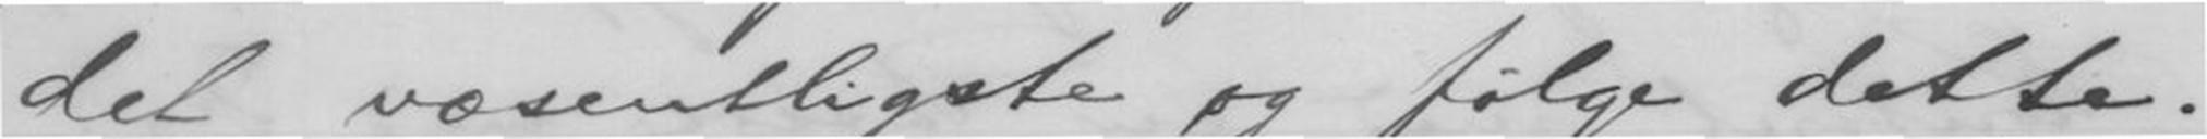det væsentligste og følge dette.
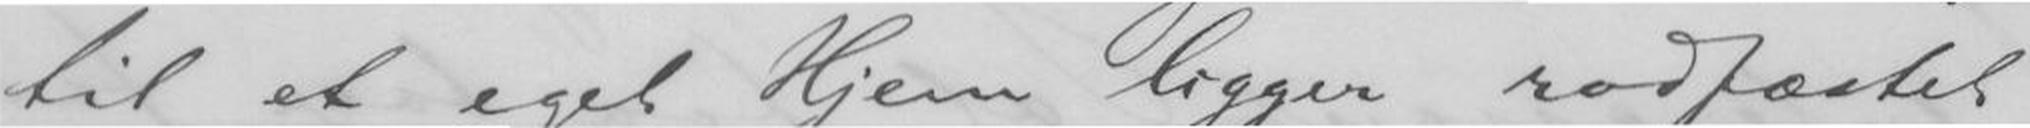til et eget Hjem ligger rodfæstet
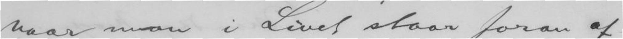naar man i Livet staar foran af-
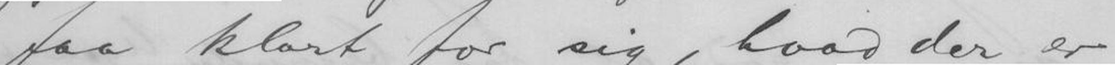faa klart for sig, hvad der er
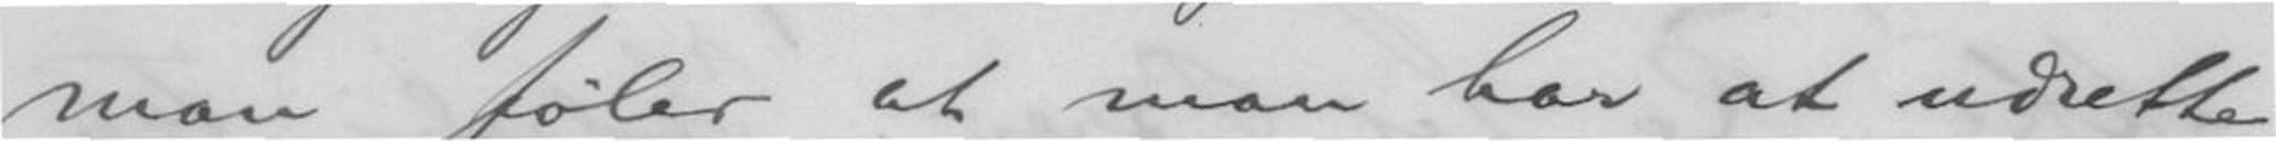man føler at man har at udrette
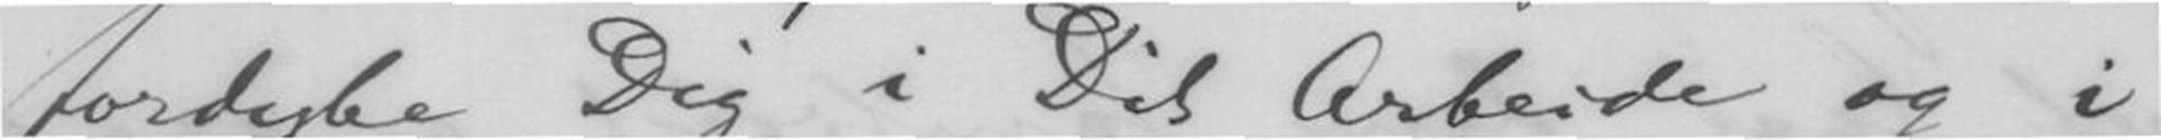fordybe Dig i Dit Arbeide og i
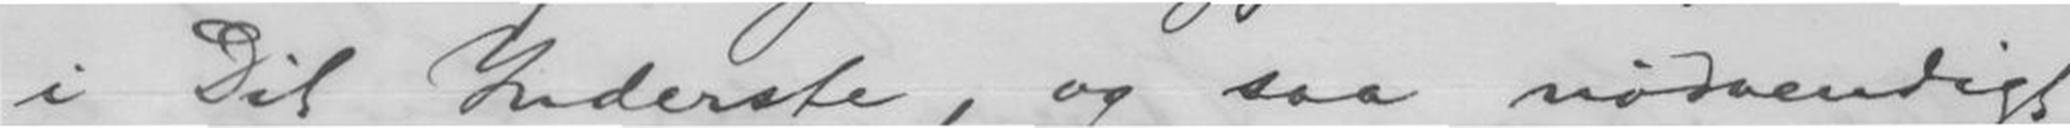i Dit Inderste, og saa nødvendigt
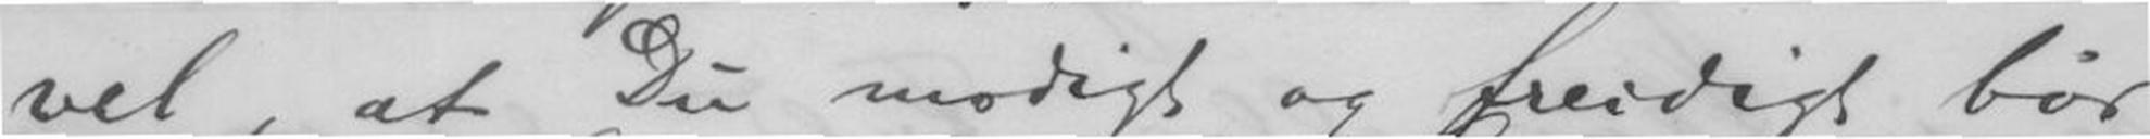vel, at Du modigt og freidigt bør
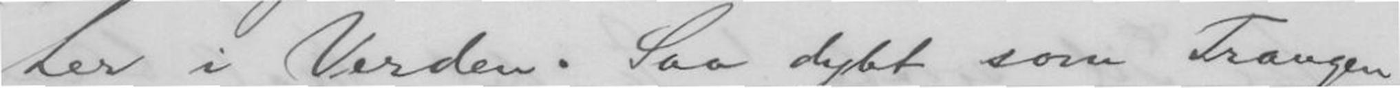her i Verden. Saa dybt som Trangen
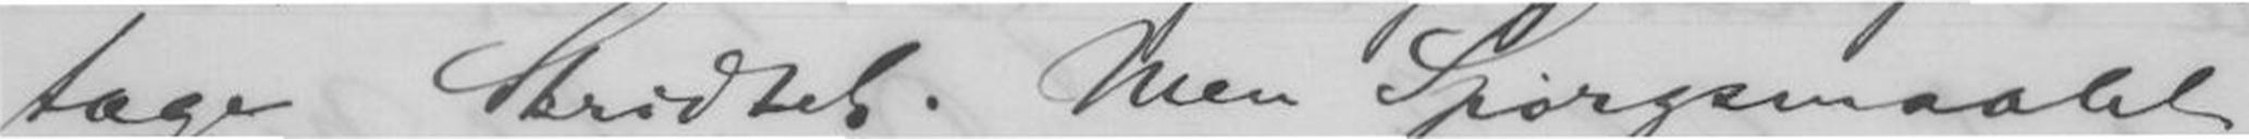tage Skridtet. Men Spørgsmaalet
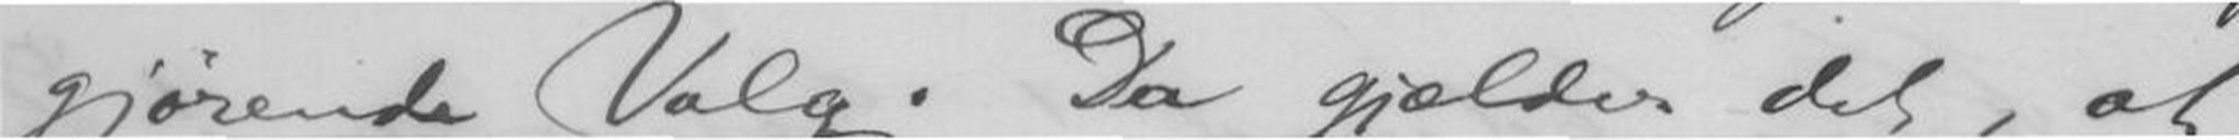gjørende Valg. Da gjælder det, at
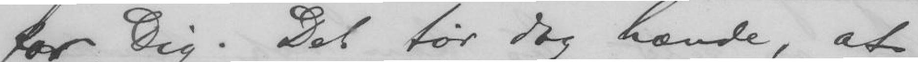for Dig. Det tør dog hænde, at
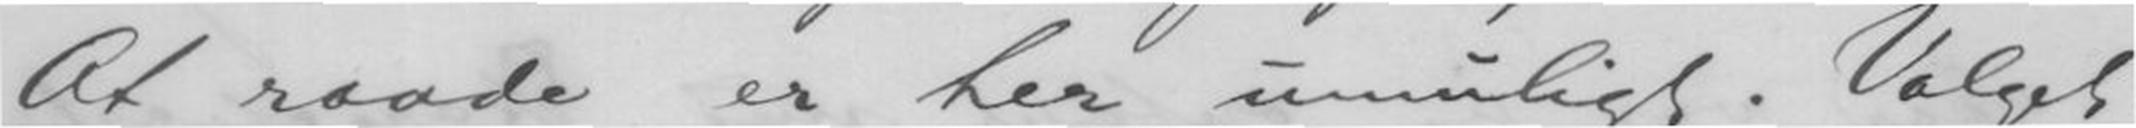At raade er her umuligt. Valget
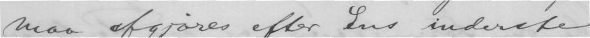maa afgjøres efter Ens inderste
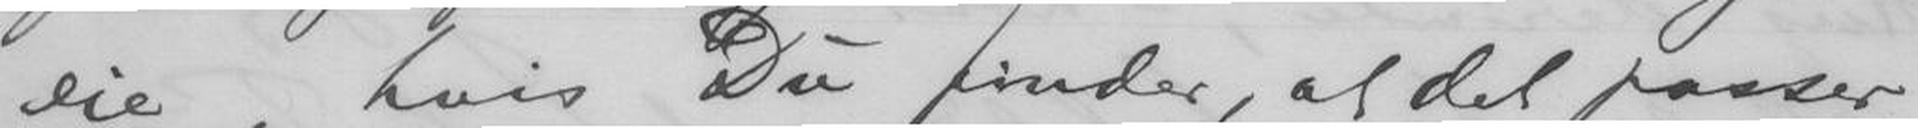eie, hvis Du finder, at det passer
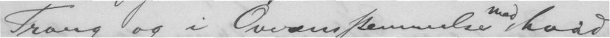Trang og i Overænsstemmelse hvad
